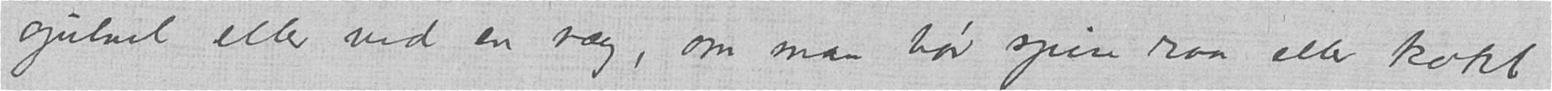gulvet eller ved en væg, om man bør spise raa eller kokt
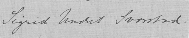Sigrid Undset Svarstad.
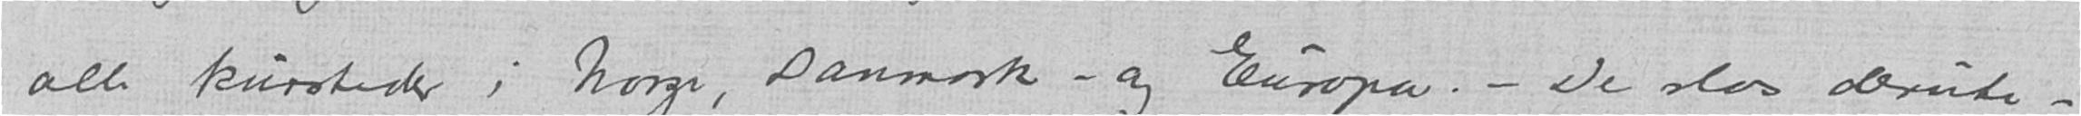alle kursteder i Norge, Danmark – og Europa. – De slos derute –
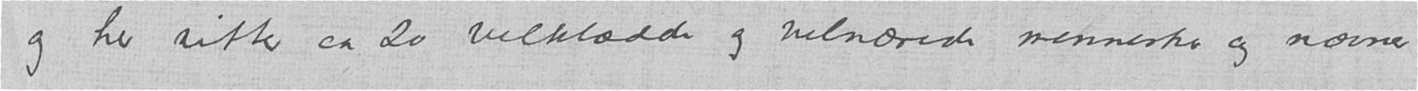og her sitter ca 20 velklædde og velnærede mennesker og nævner
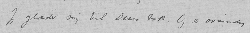Jeg glæder mig til Deres bok. Og er avsindig
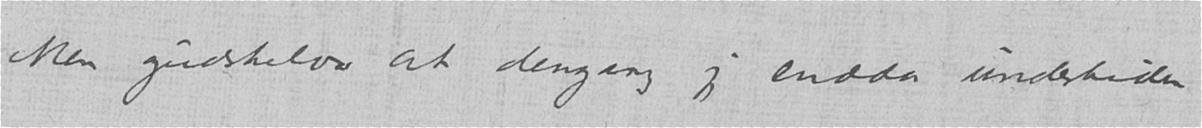Men gudskelov at dengang jeg endda undertiden
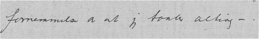fornemmelse av at jeg taaler alting – .
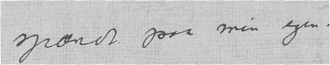spændt paa min egen.
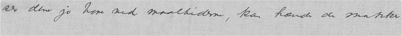ser dem jo bare ved maaltiderne, kan hænde de snakker
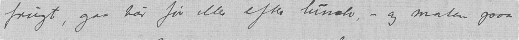frugt, gaa tur før eller efter lunch, - og maten paa
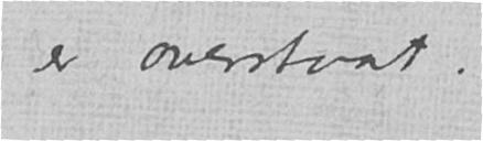er overstaat.
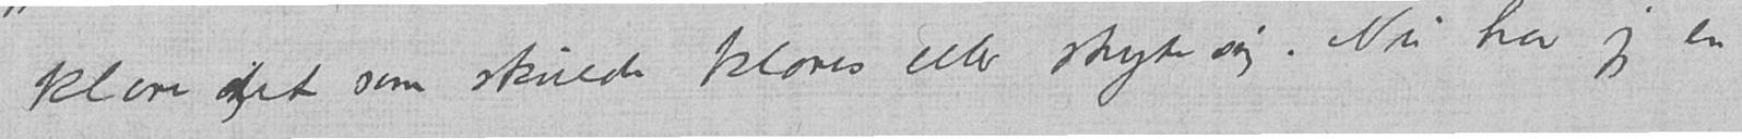klare det som skulde klares eller skyte sig. Nu har jeg en
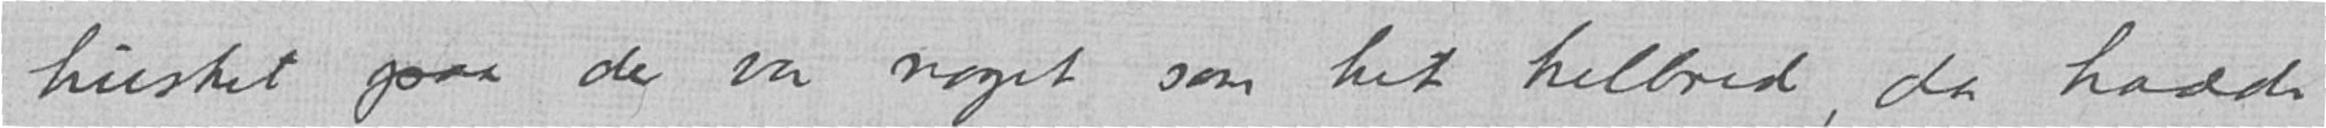husket paa der var noget som het helbred, da hadde
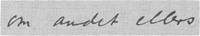om andet ellers
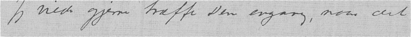Jeg vilde gjerne træffe Dem engang, naar det
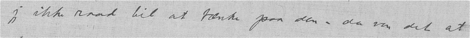jeg ikke raad til at tænke paa den - da var det at
